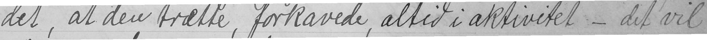det at den trætte, forkavede, altid i aktivitet - det vil
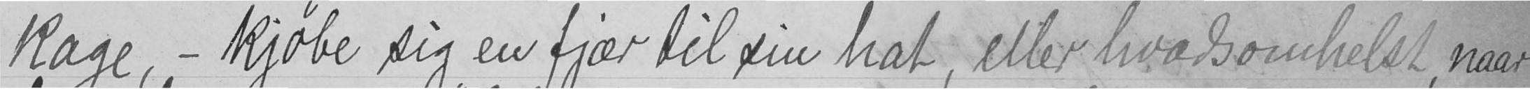kage, - kjøbe sig en fjær til sin hat, eller hvadsomhelst, naar
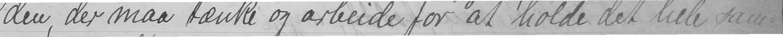den, der maa tænke og arbeide for "at holde det hele sam-
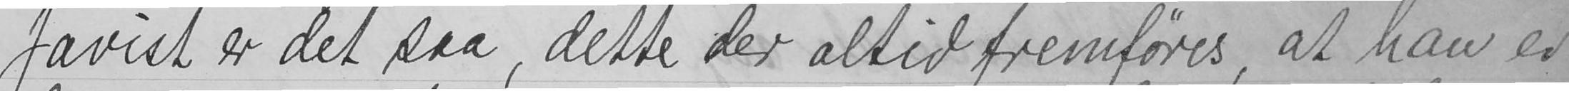Javist er det saa, dette der altid fremføres, at han er
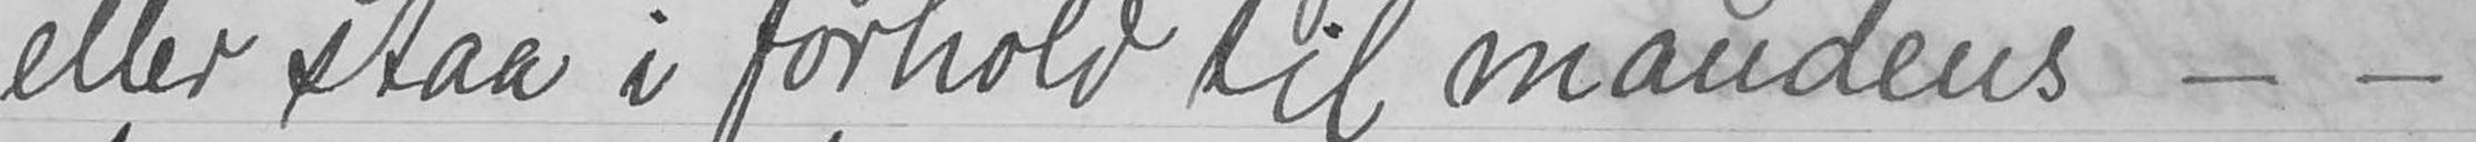eller staa i forhold til mandens - -
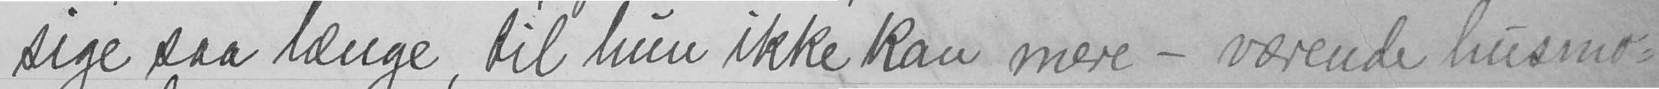sige saa længe, til hun ikke kan mere - værende husmo-
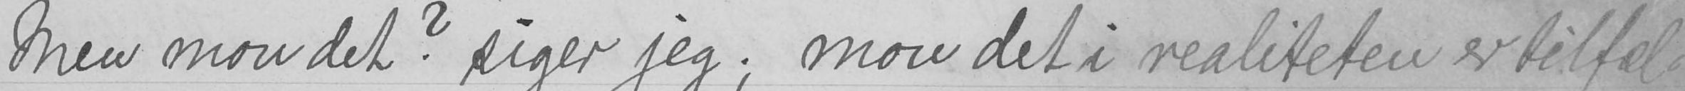Men mon det? siger jeg; mon det i realiteten er tilfel-
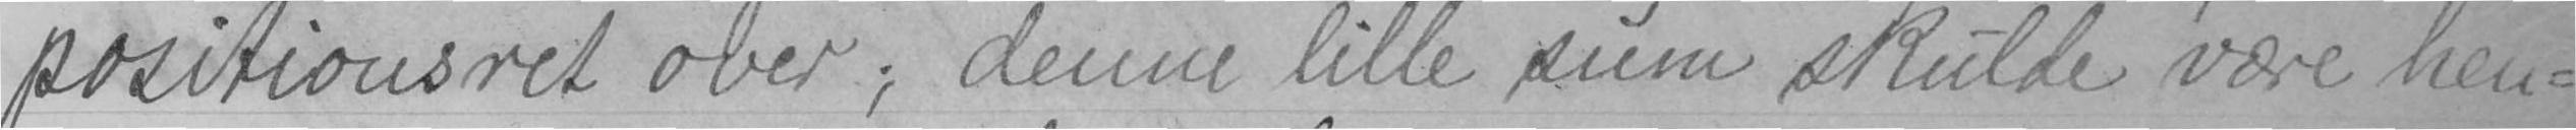positionsret over; denne lille sum skulde være hen-
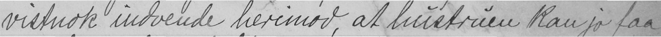vistnok indvende herimod, at hustruen kan jo faa
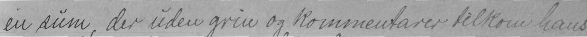en sum, der uden grin og kommentarer tilkom hans
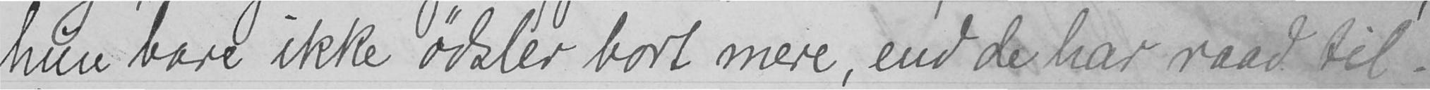hun bare ikke ødsler bort mere, end de har raad til.
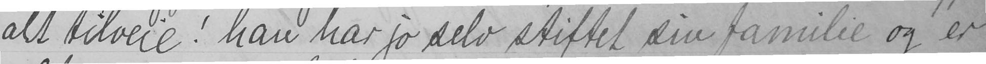alt tilveie! han har jo selv stiftet sin familie og er
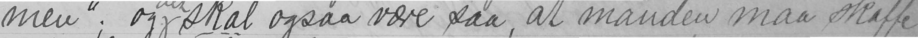men"; og det skal ogsaa være saa, at manden maa skaffe
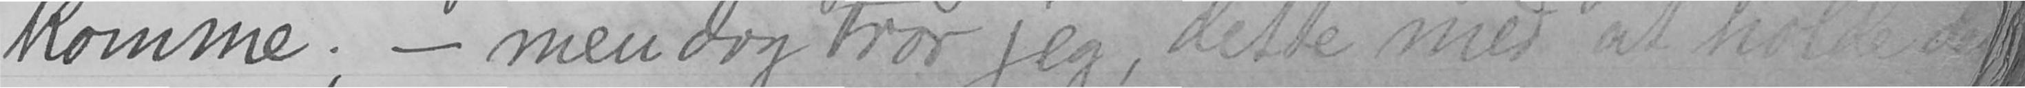komne; - men dog tror jeg, dette med "at holde det
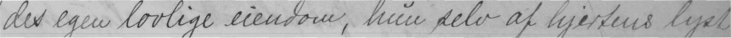des egen lovlige eiendom, hun selv af hjertens lyst
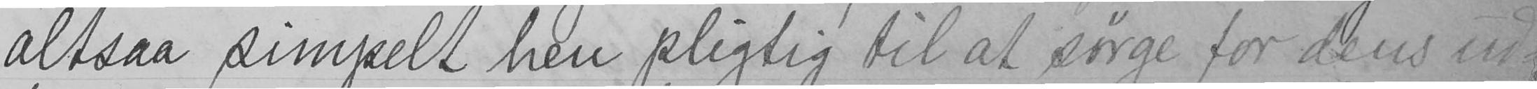altsaa simpelt hen pligtig til at sørge for dens ind-
